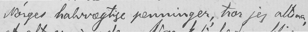Norges halvvægtige penninger, tror jeg altsaa,
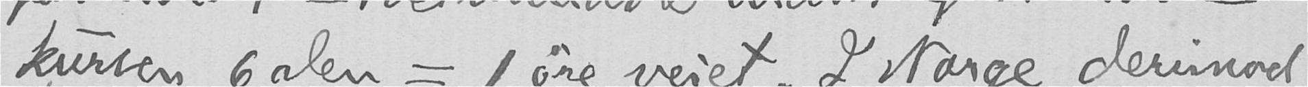kursen 6 alen = 1 øre veiet. I Norge derimod
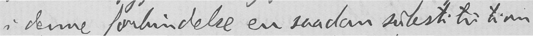i denne forbindelse en saadan substitution
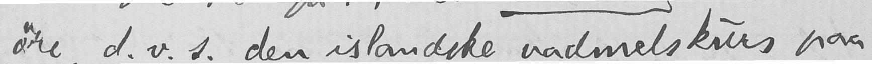øre, d. v. s. den islandske vadmelskurs paa
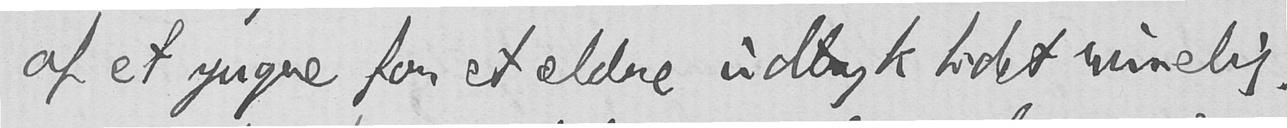af et yngre for et ældre udtryk lidet rimelig.
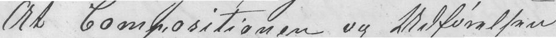At Compositionen og Udførelsen
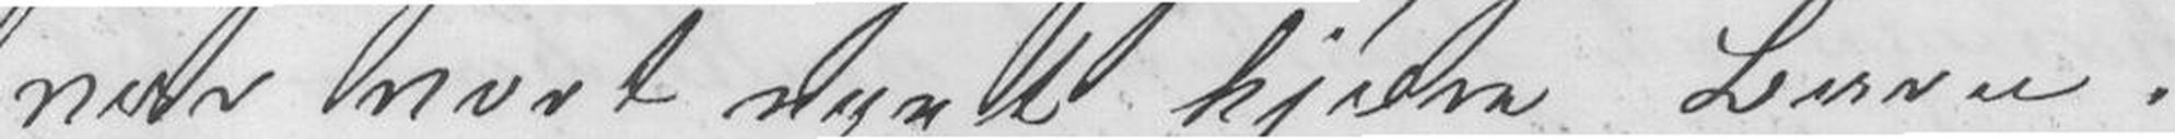var vort eget kjære Barn.
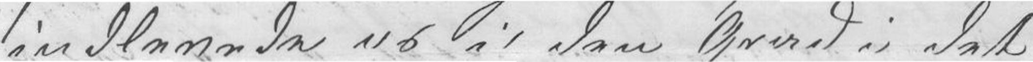indlevede os i den Grad det
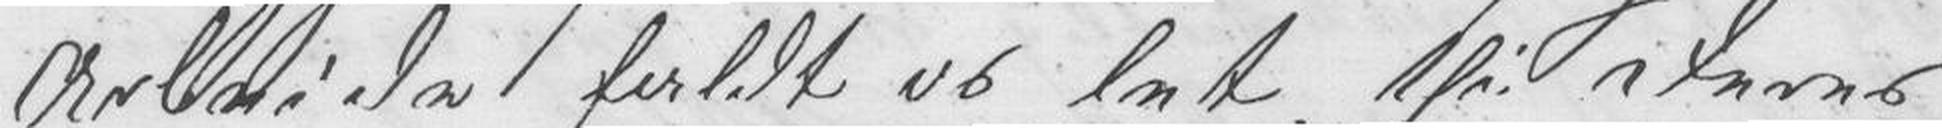Arbeide faldt os let thi Deres
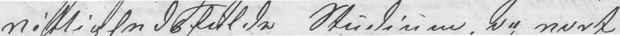vittighedsfulde Studium og vort
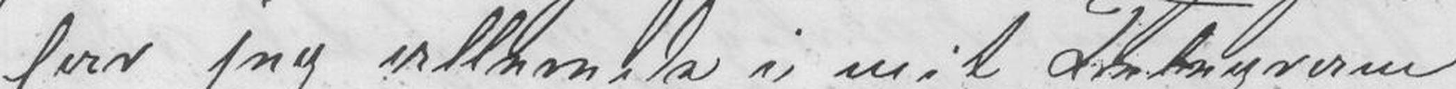har jeg allerede i mit Telegram
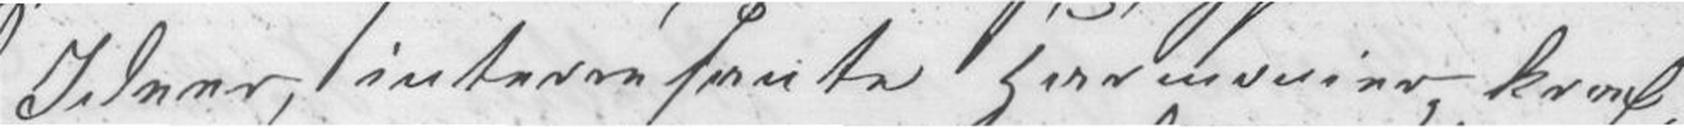Ideer, interresante Harmonier kraf-
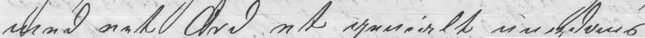med eet Ord, et genialt ungdoms-
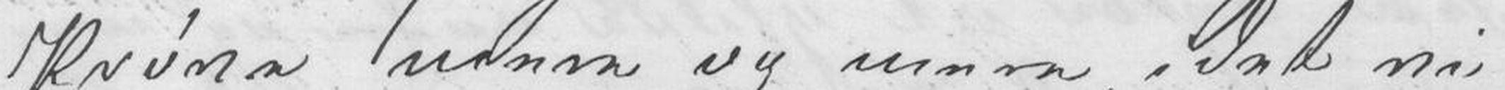Prøve mere og mere, idet vi
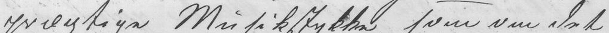prægtige Musikstykke, som om det
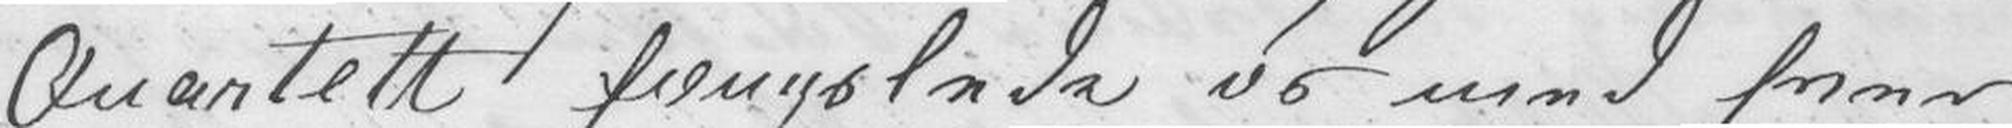Quartett fængslede os med hver
